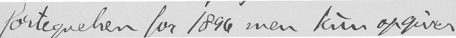fortegnelsen for 1896, men kun opgiver
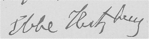Ebbe Hertzberg
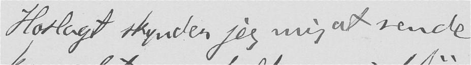Hoslagt skynder jeg mig at sende
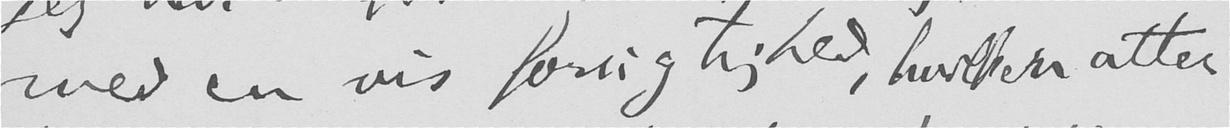med en vis forsigtighed, hvilken atter
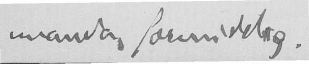mandag formiddag.
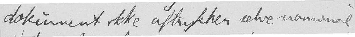dokument ikke aftrykker selve nominal-
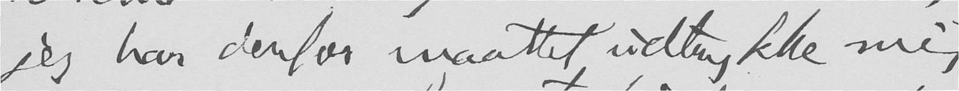jeg har derfor maattet udtrykke mig
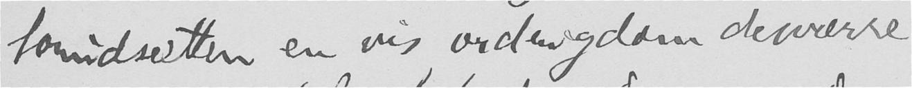forudsætter en vis ordrigdom desværre.
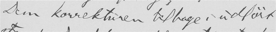Dem korrekturen tilbage i udført
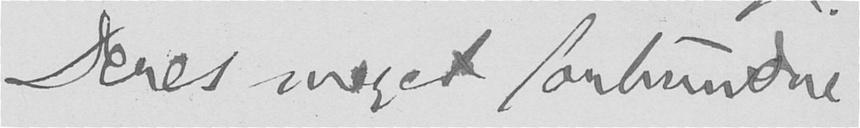Deres meget forbundne
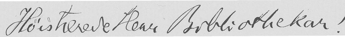Høistærede Herr Bibliothekar!
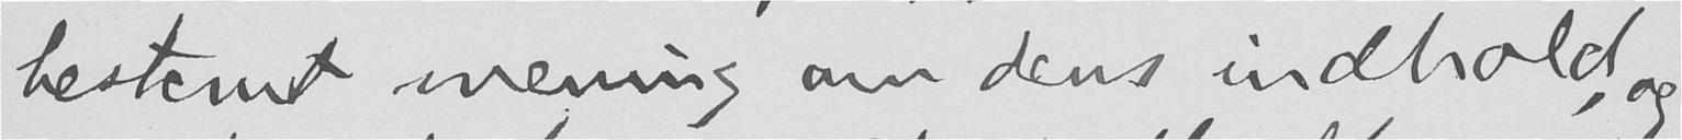bestemt mening om dens indhold, og
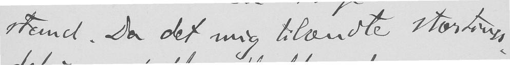stand. Da det mig tilsendte stortings-
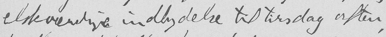elskværdige indbydelse til tirsdag aften,
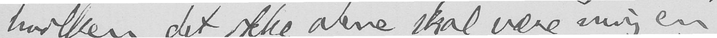hvilken det ikke alene skal være mig en
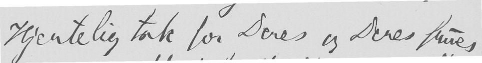Hjertelig tak for Deres og Deres frues
